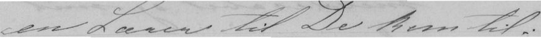en Lærer til De kom til-
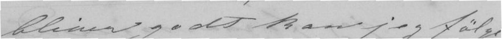bliver godt kan jeg følge
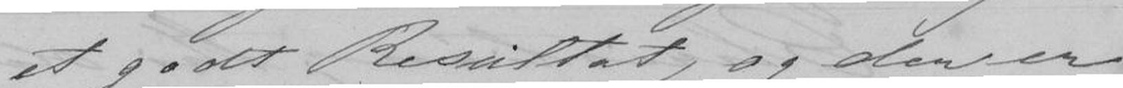et godt Resultat, og der er
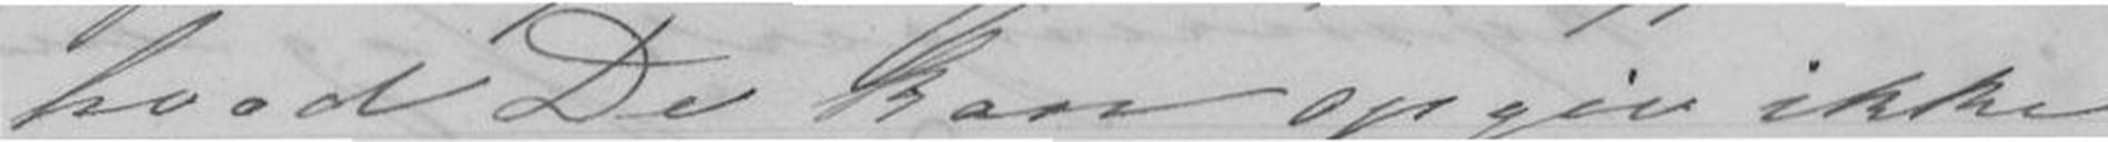hvad De kan opgiv ikke
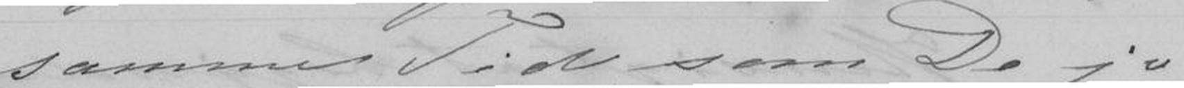samme tid som De jo
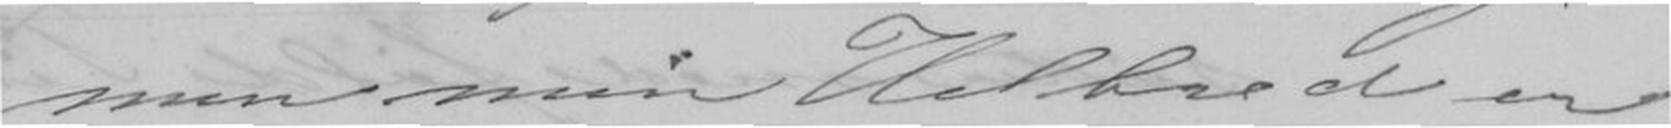men min Helbred er
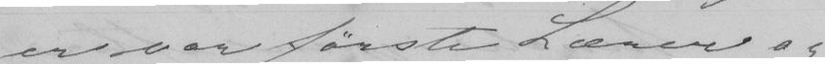er vor første Lærer og
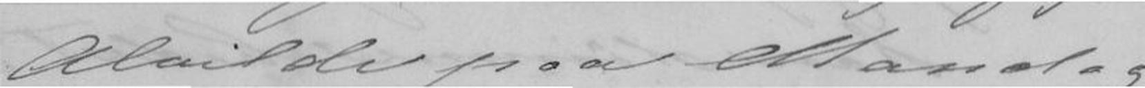Alvilde paa Mandag
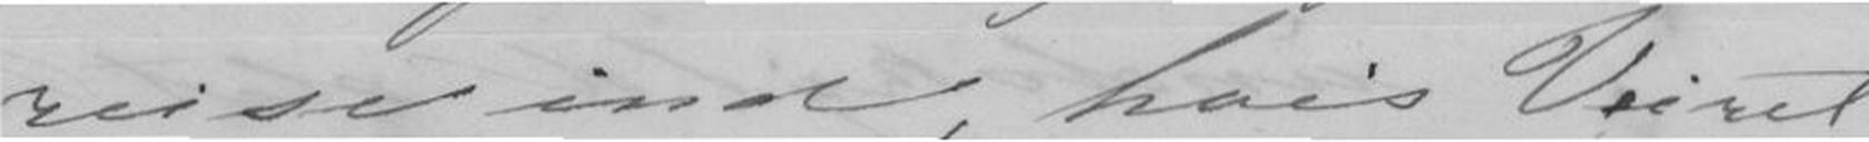reise ind, hvis Veiret
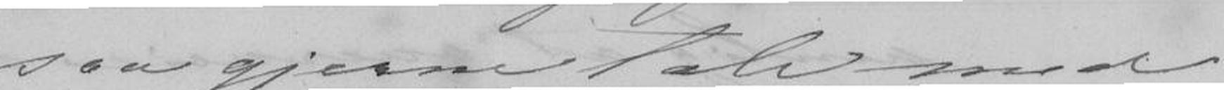saa gjerne tale med
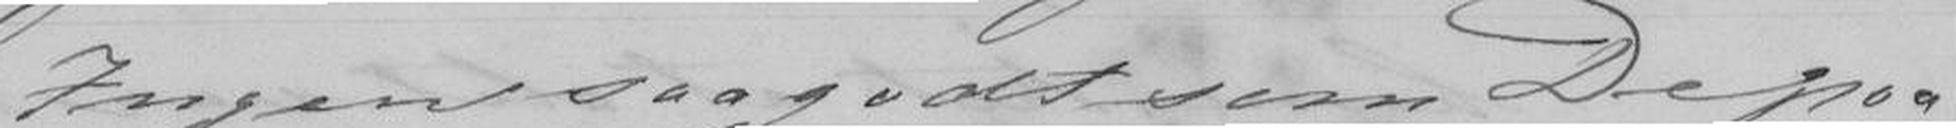Ingen saa godt som De paa
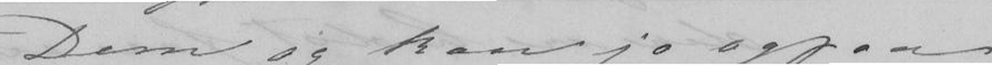Dem og kan jo ogsaa
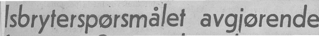Isbryterspørsmålet avgjørende
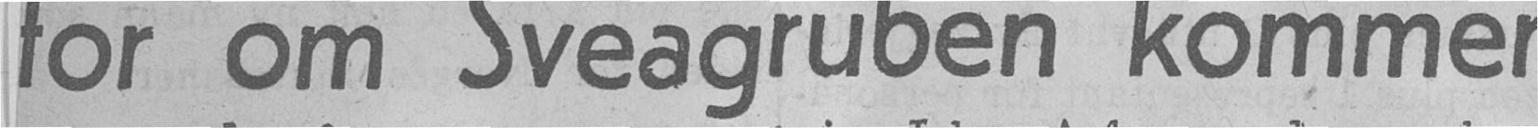for om Sveagruben kommer
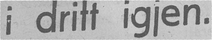i drift igjen.
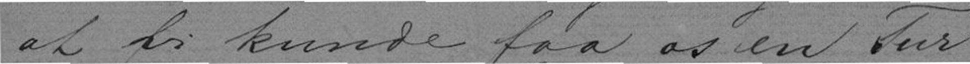at vi kunde faa os en Tur
